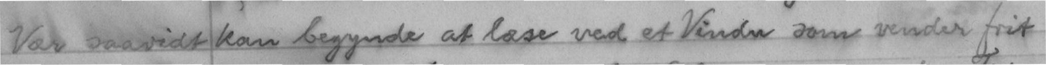Vær saavidt kan begynde at læse ved et Vindu som vender frit
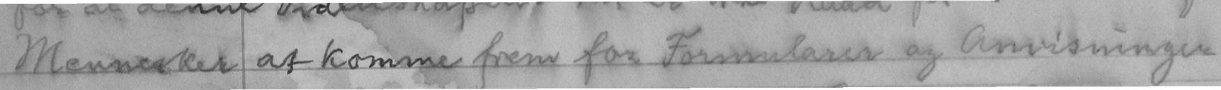Mennesker at komme frem for Formularer og Anvisninger
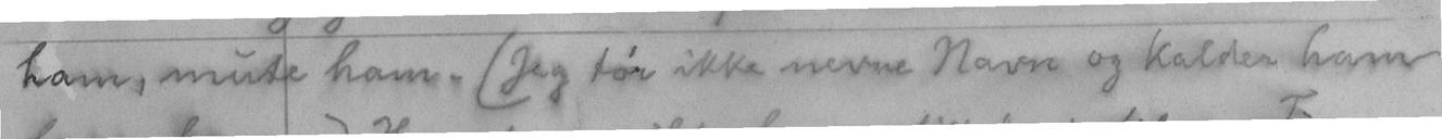ham, mute ham. (Jeg tør ikke nevne Navn og kalder ham
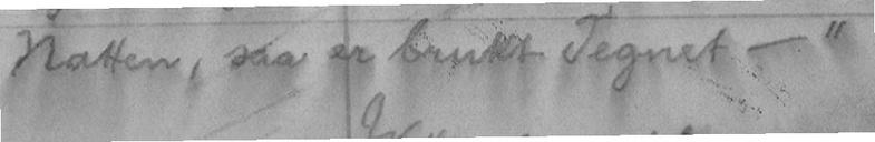Natten, saa er brukt Tegnet - "
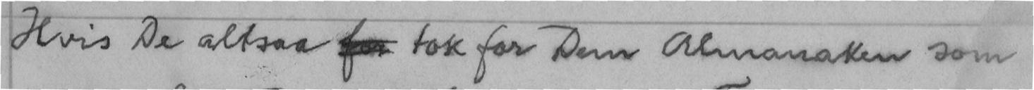Hvis De altsaa for tok for Dem Almanaken som
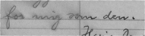for mig som den.
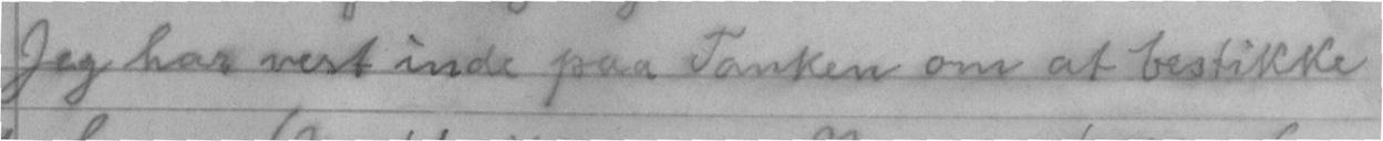Jeg har vert inde paa Tanken om at bestikke
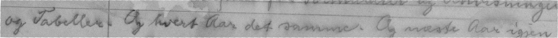og Tabeller. Og hvert Aar det samme. Og næste Aar igjen
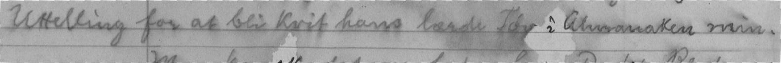Uttelling for at bli kvit hans lærde Tøv i Almanaken min.
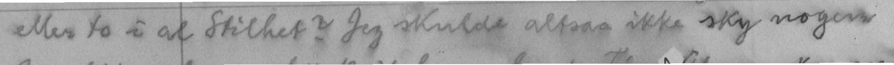eller to i al Stilhet? Jeg skulde altsaa ikke sky nogen
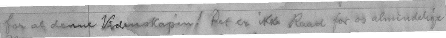for al denne Videnskapen! Det er ikke Raad for os almindelige
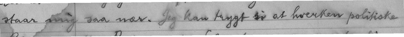staar mig saa nær. Jeg kan trygt si at hverken politiske
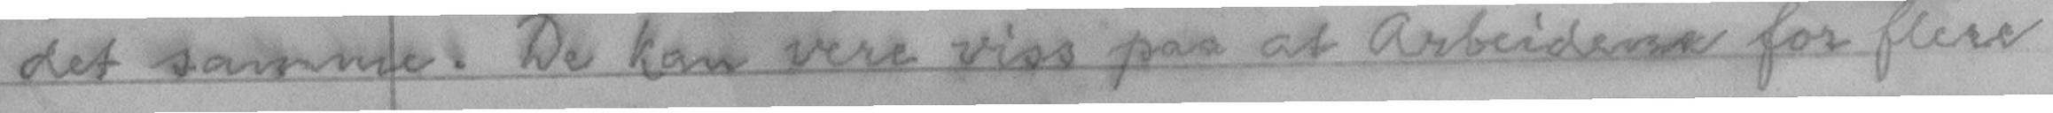det samme. De kan vere viss paa at Arbeiderne for flere
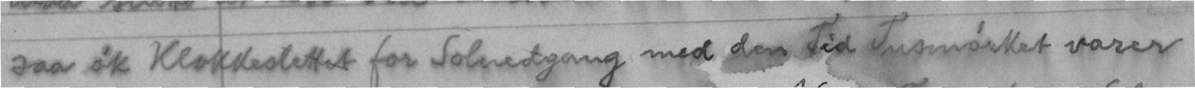saa øk Klokkeslettet for Solnedgang med den Tid Tusmørket varer
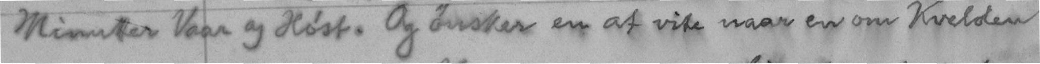Minutter Vaar og Høst. Og ønsker en at vite naar en om Kvelden
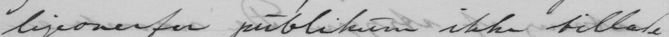ligeoverfor publikum ikke tillade
